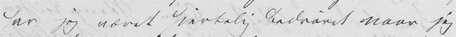har jeg været hjertelig bedrøvet naar jeg
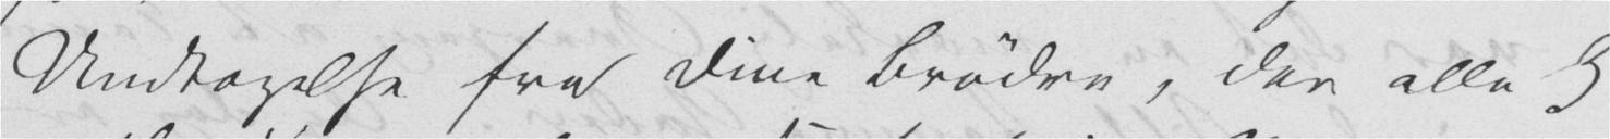Undtagelse fra Dine Brødre, der alle 3
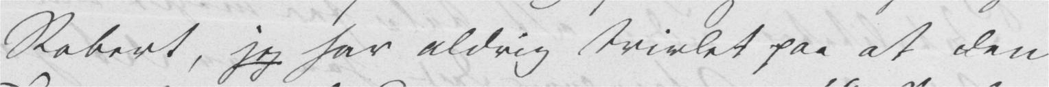Robert, jeg har aldrig tvivlet paa at den
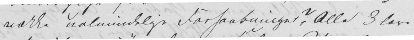vække ualmindelige Forhaabninger? Alle 3 love
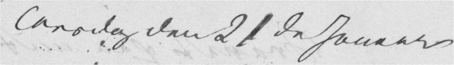Torsdag den 21de Januar
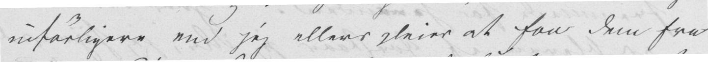udførligere end jeg ellers pleier at faa dem fra
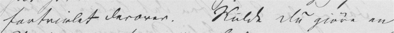fortvivlet derover. Skulde Du gjøre en
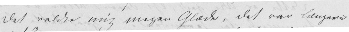det voldte mig megen Glæde, det var længere
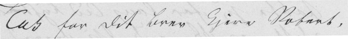Tak for Dit Brev kjere Robert,
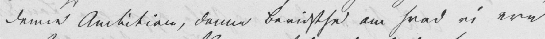denne Ambition, denne Bevidsthed om hvad vi ere
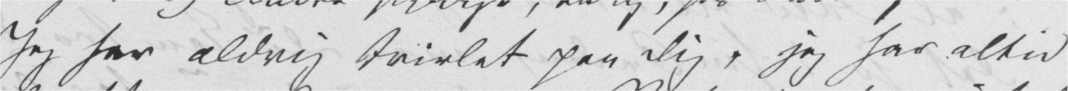Jeg har aldrig tvivlet paa Dig, jeg har altid
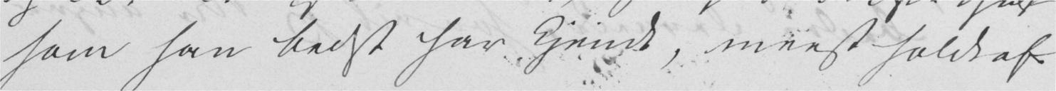som han bedst har kjendt, meest holdt af.
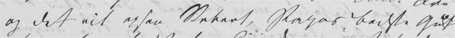og det vil ogsaa Robert, Papas bedste Gut
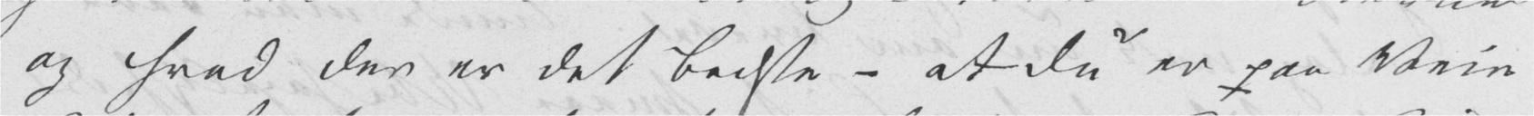og hvad der er det bedste – at Du er paa Veie
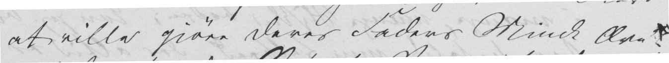at ville gjøre deres Faders Minde Ære!
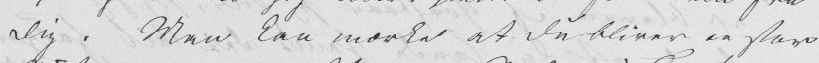Dig. Man kan mærke at Du bliver en stor
